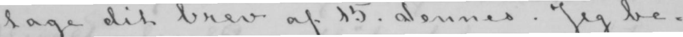tage dit brev af 15. dennes. Jeg be-
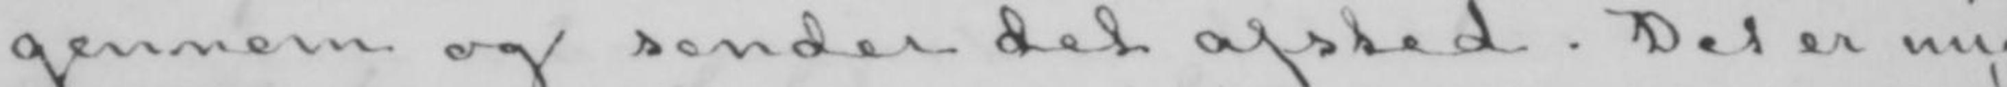gennem og sender det afsted. Det er mig
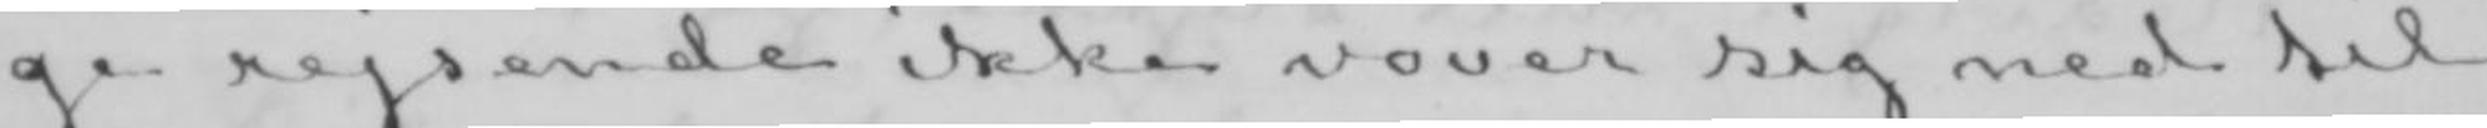ge rejsende ikke vover sig ned til
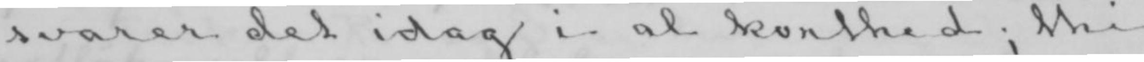svarer det idag i al korthed; thi
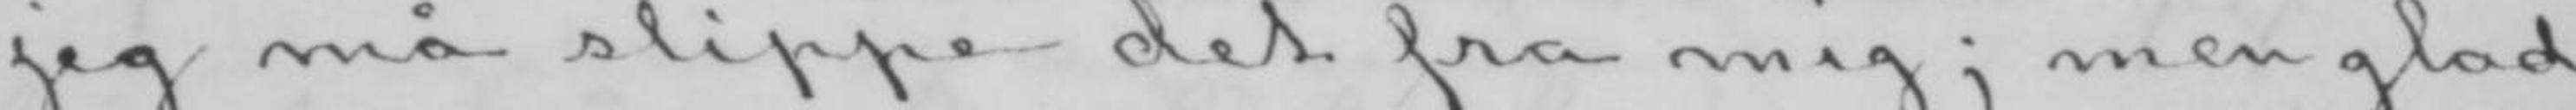jeg må slippe det fra mig; men glad
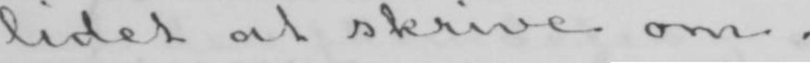lidet at skrive om.
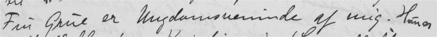Fru Grue er Ungdomsveninde at mig. Hun er
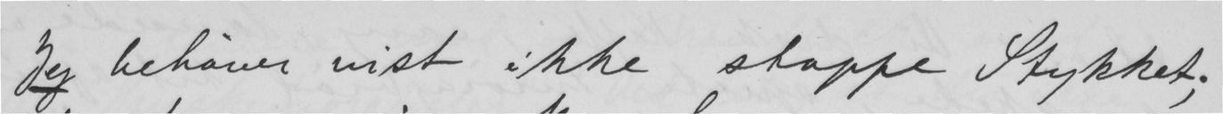Jeg behøver vist ikke stoppe Stykket;
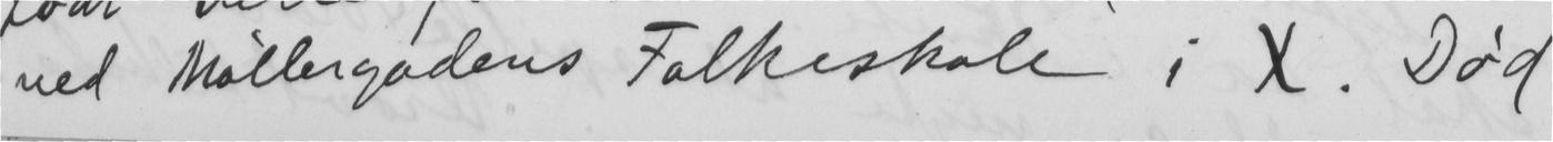ved Møllergadens Folkeskole i X. Død
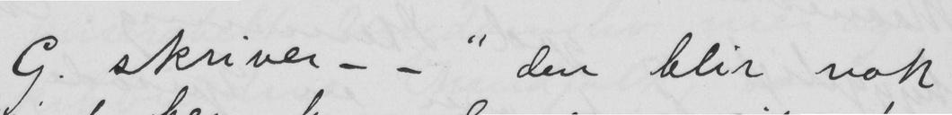G. skriver - - "den blir nok
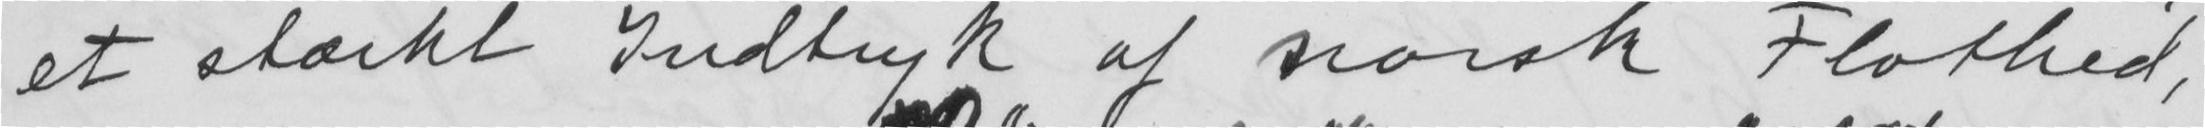et stærkt Indtryk af norsk Flothed,
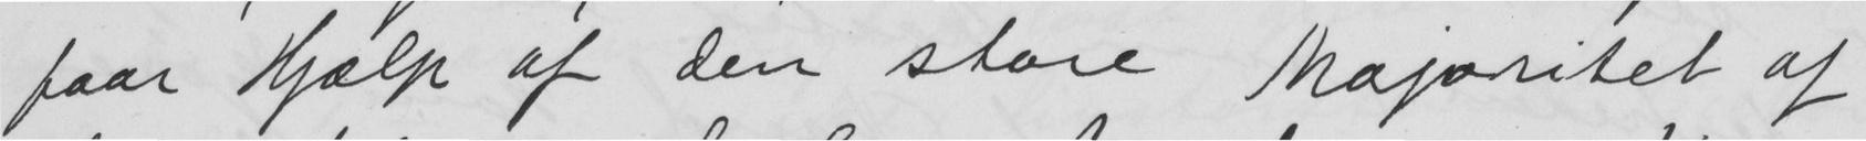faar Hjælp af den store Majoritet af
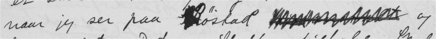naar jeg ser paa Røstad  og
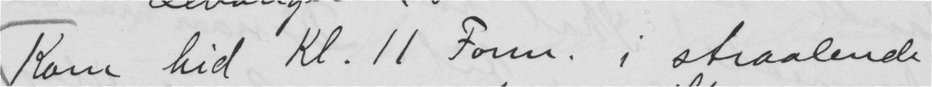Kom hid Kl. 11 Form. i straalende
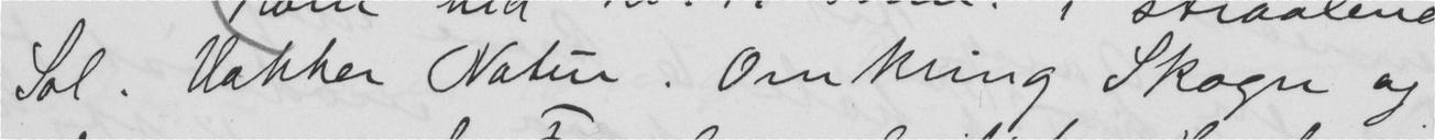Sol. Vakker Natur. Omkring Skogn og
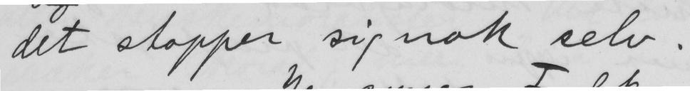det stopper sig nok selv.
